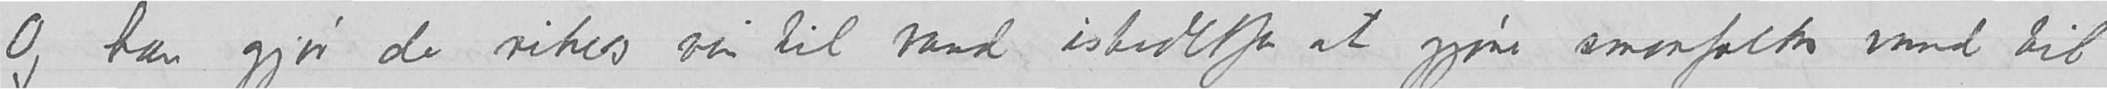Og han gjør de rikes vin til vand istedetfor at gjøre smaafolks vand til vin.
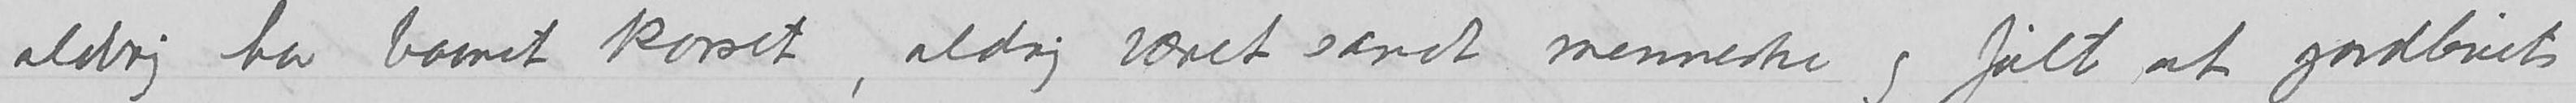aldrig har baaret korset, aldrig været sandt menneske og følt at jordlivets
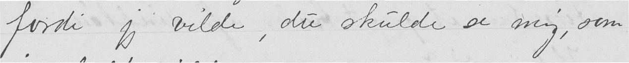fordi jeg vilde, du skulde se mig, som
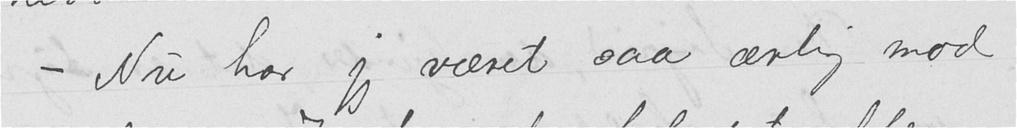- Nu har jeg været saa ærlig mod
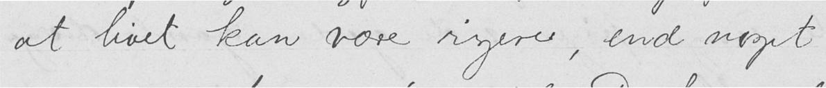at livet kan være rigere, end noget
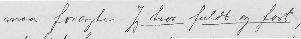maa foragte. Jeg tror fuldt og fast,
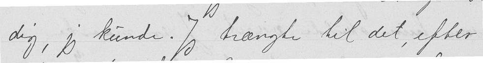dig, jeg kunde. Jeg trængte til det, efter
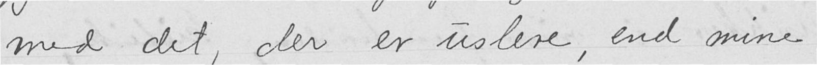med det, der er uslere, end mine
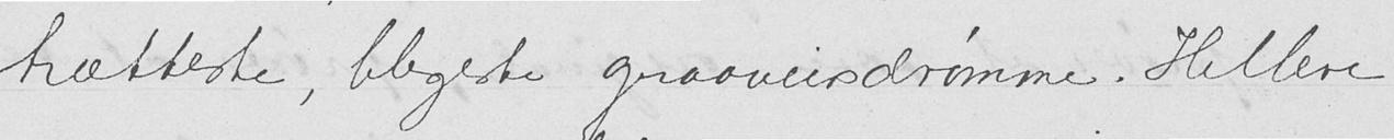trætteste, blegeste graaveirsdrømme. Hellere
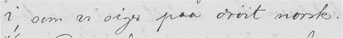i, som vi siger paa drøit norsk.
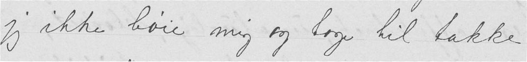jeg ikke nøie mig og tage til takke
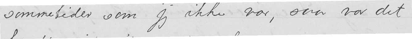sommetider som jeg ikke var, saa var det
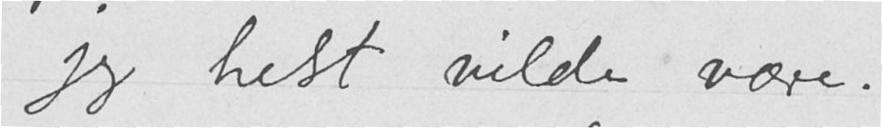jeg helst vilde være.
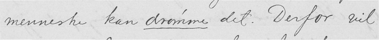menneske kan drømme det. Derfor vil
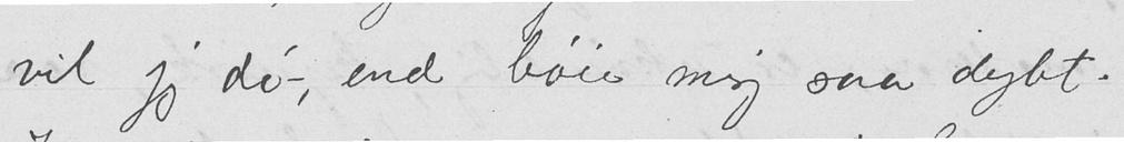vil jeg dø, end bøie mig saa dybt.
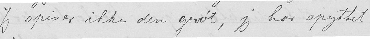Jeg spiser ikke den grøt, jeg har spyttet
